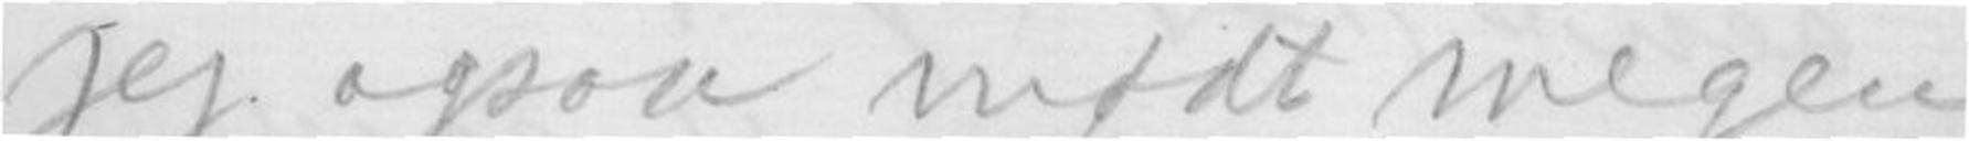har jeg ogsaa mødt megen
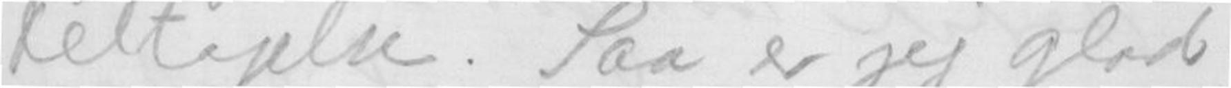Deltagelse. Saa er jeg glad
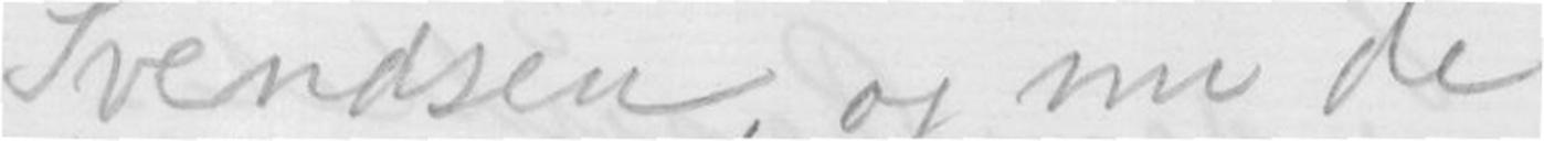Svendsen, og nu de
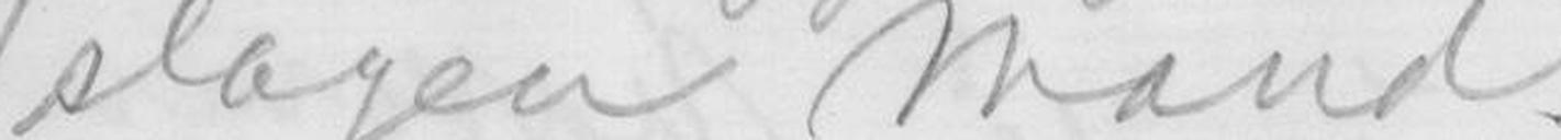slagen Mand.
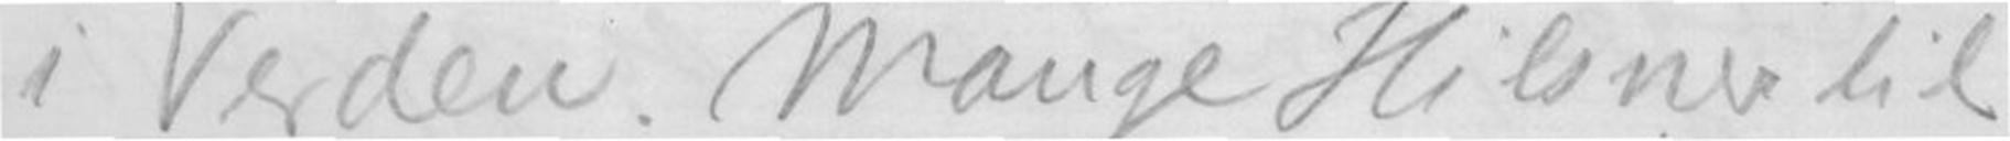i Verden. Mange Hilsener til
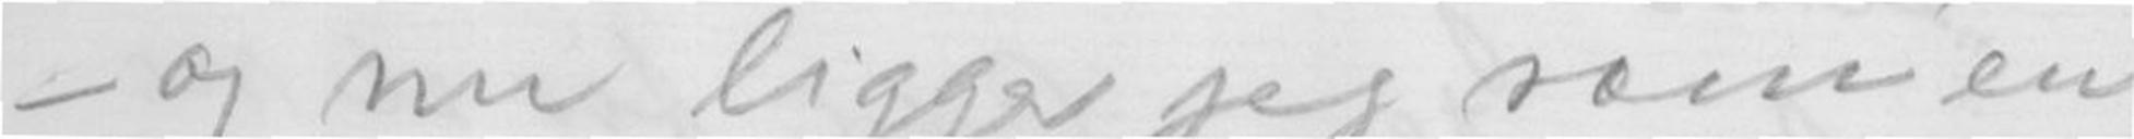- og nu ligger jeg som en
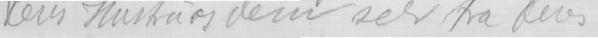Deres Hustru og Dem selv fra Deres
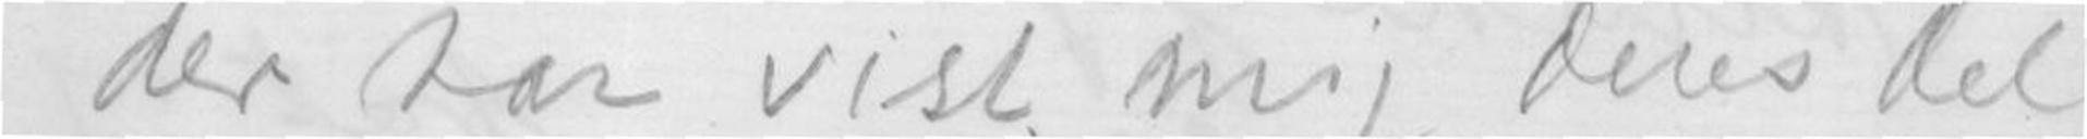der har vist mig Deres Del-
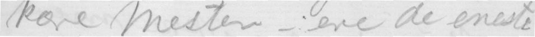kære Mester - ere de eneste
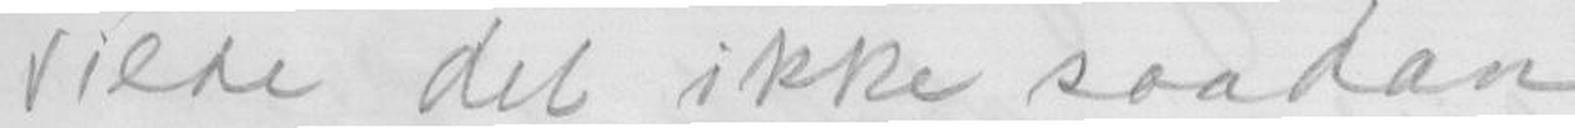vilde det ikke saadan,
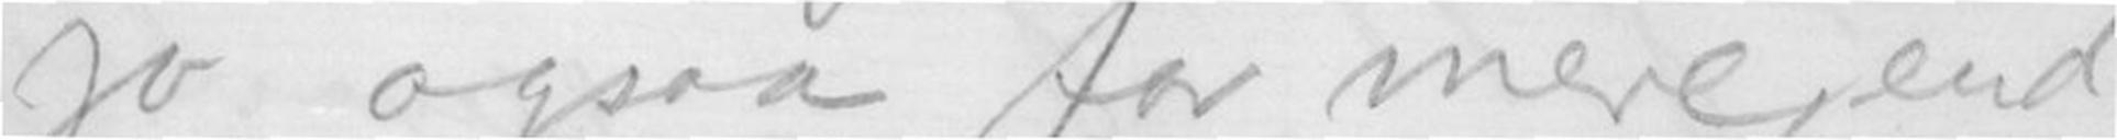jo ogsaa for mere, end
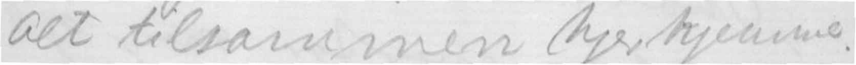alt tilsammen hjer hjemme.
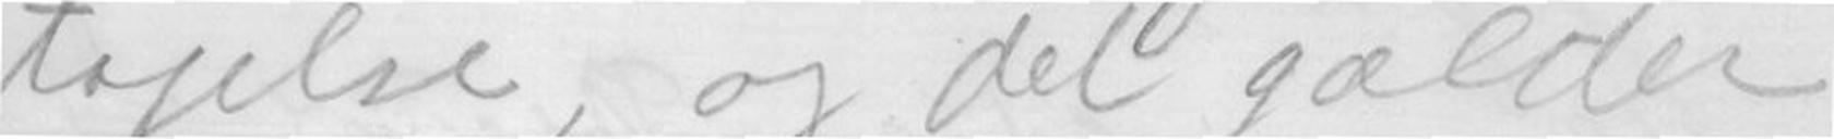tagelse, og det gælder
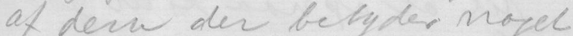af dem der betyder noget
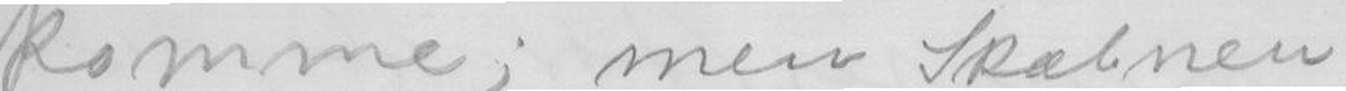komme; men Skæbnen
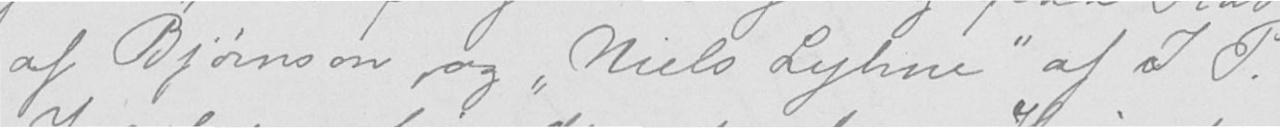af Bjørnson og "Niels Lyhne" af J. P.
